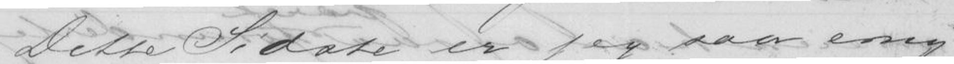Dette Sidste er jeg saa enig
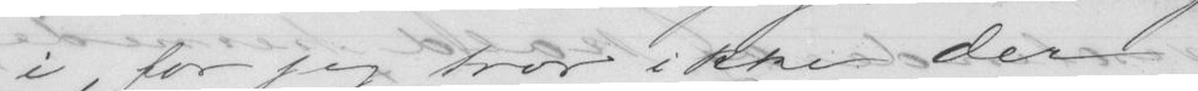i, for jeg tror ikke der
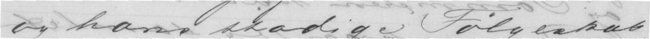og hans stadige Følgeskab,
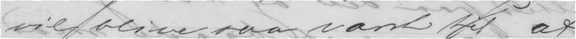vil blive saa vant til at
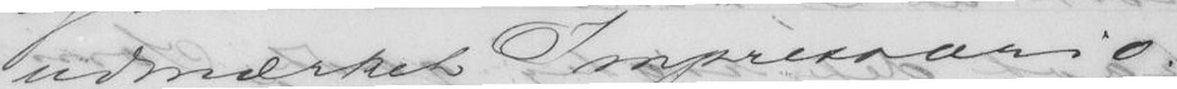udmærket Impressario.
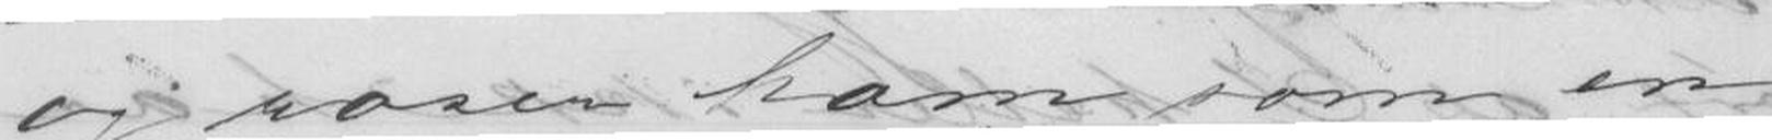og roser ham som en
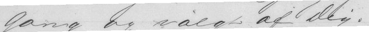Gang og valgt af Dig.
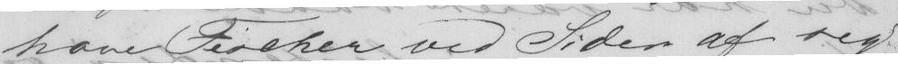have Fischer ved Siden af sig,
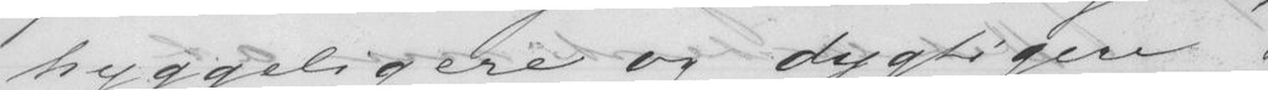hyggeligere og dygtigere
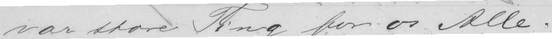var store Ting for os Alle;
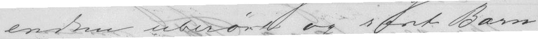endnu uberørt og rent Barn
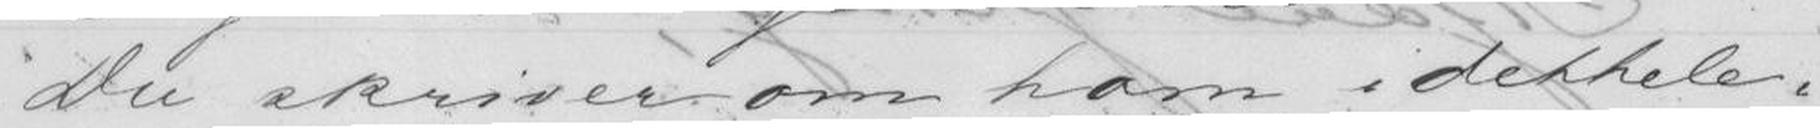Du skriver om ham i dethele-
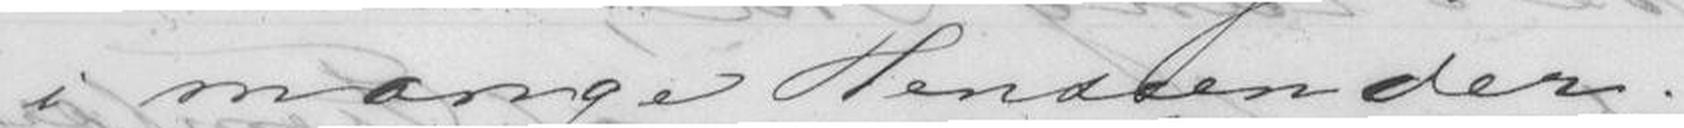i mange Henseender.
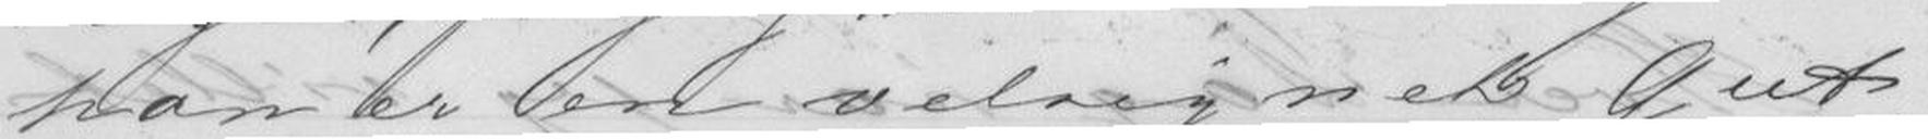han er en velsignet Gut
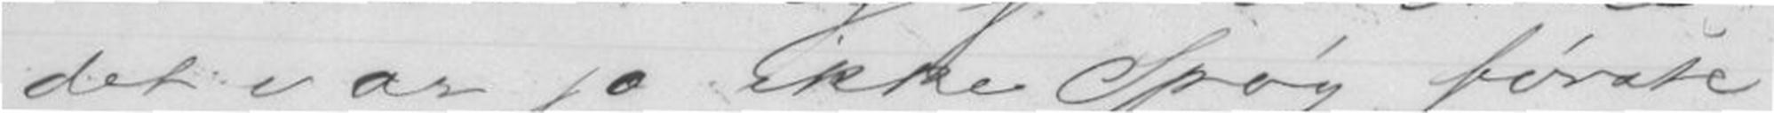det var jo ikke Spøg første
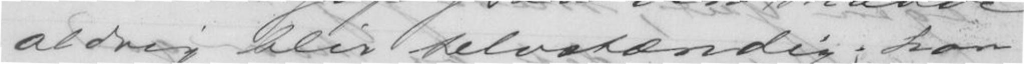aldrig blir selvstændig; han
